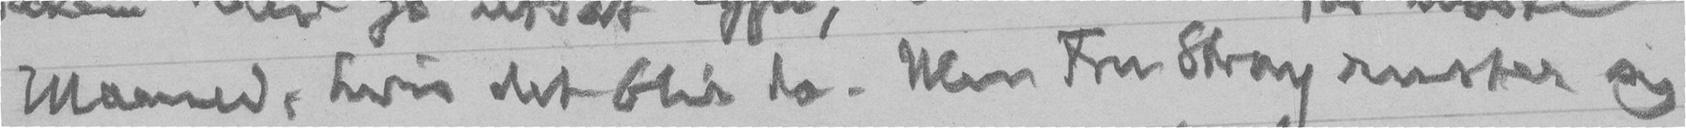Maaned, hvis det blir da. Men Fru Stray ruster sig
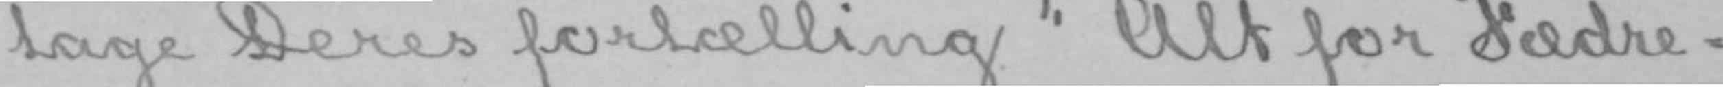tage Deres fortælling «Alt for Fædre-
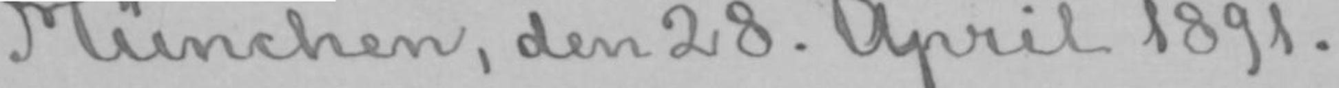München, den 28. April 1891.
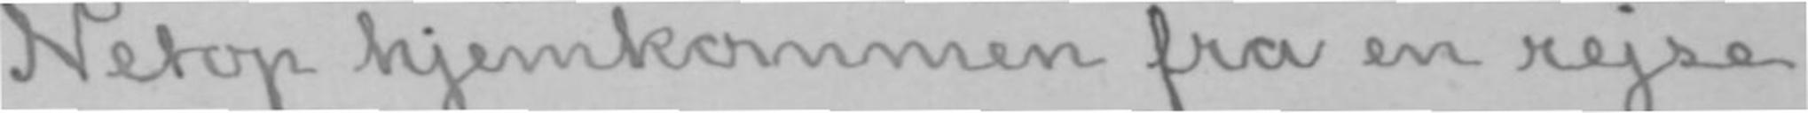Netop hjemkommen fra en rejse
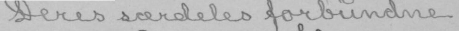Deres særdeles forbundne
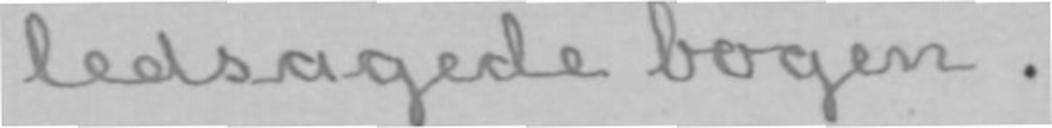ledsagede bogen.
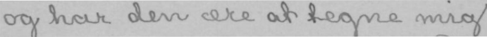og har den ære at tegne mig
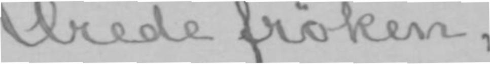Ærede frøken,
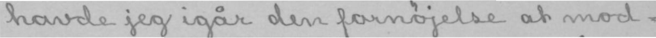havde jeg igår den fornøjelse at mod-
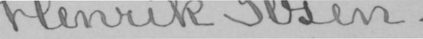Henrik Ibsen.
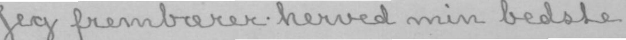Jeg frembærer herved min bedste
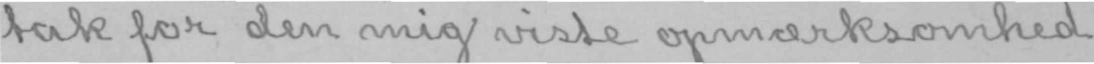tak for den mig viste opmærksomhed
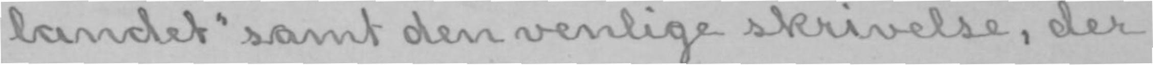landet» samt den venlige skrivelse, der
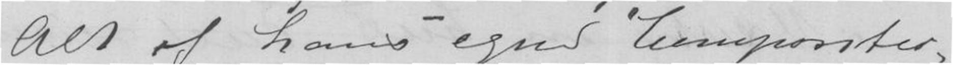Alt af hans egne Compositer-
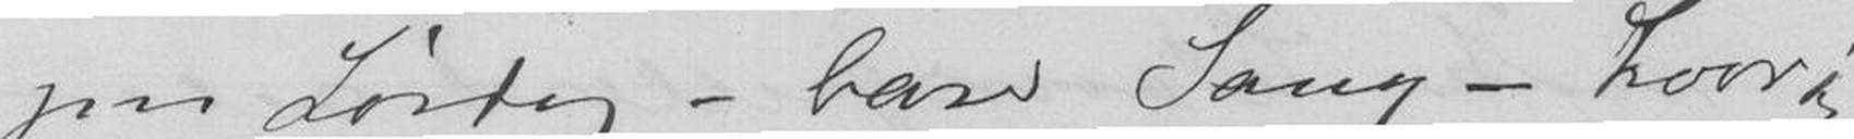paa Lørdag - bare Sang - hvori-
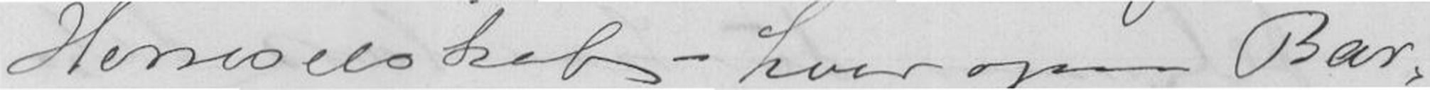Herreselskab - hvor ogsaa Bar-
